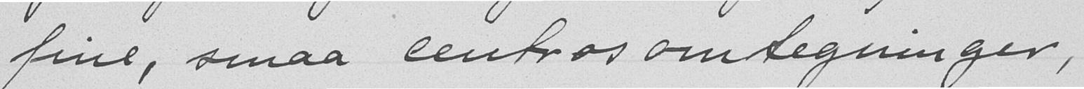fine, smaa centrøs omtegninger,
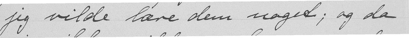jeg vilde lære dem noget; og da
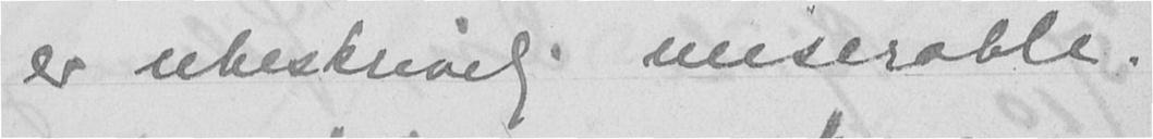er ubeskrivelig miserable.
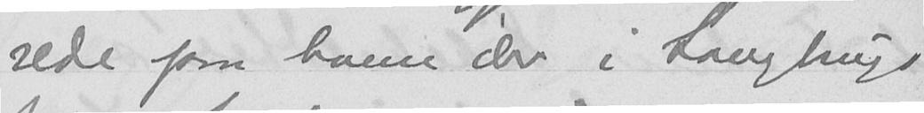rede paa hvem der i Saugbrugs-
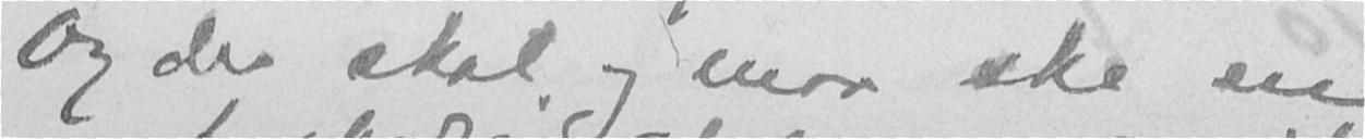Og der skal og maa ske en
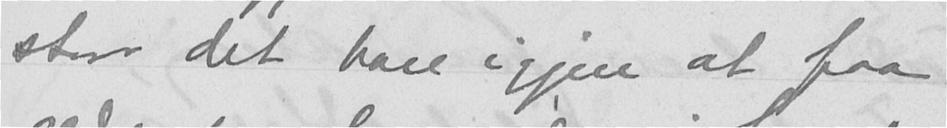staar det bare igjen at faa
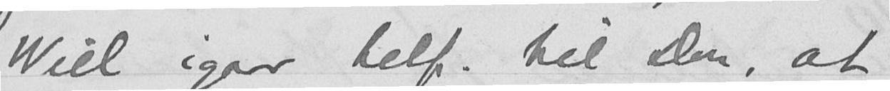Wiel igaar telf. til Don, at
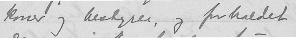koner og bestyrer, og forholdet
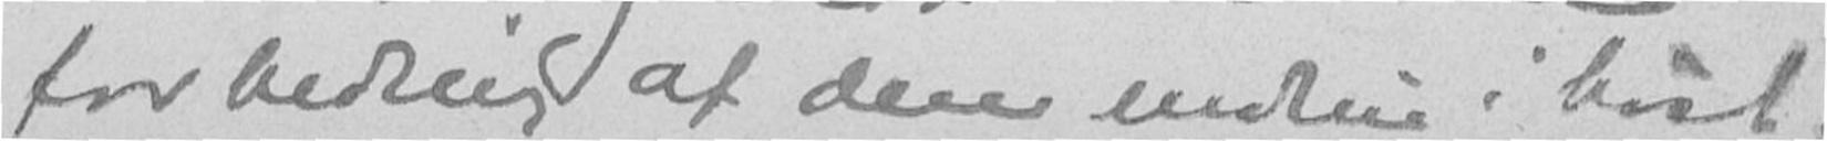forbedring af dem endnu i høst.
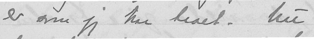er som jeg har troet. Nu
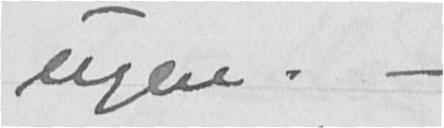ugen. -
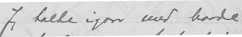Jeg talte igaar med baade
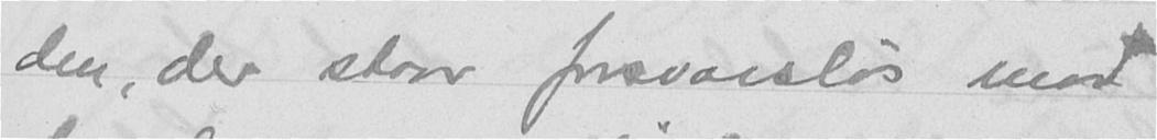den, der staar forsvarsløs mod
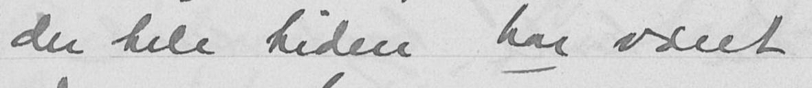der hele tiden har været
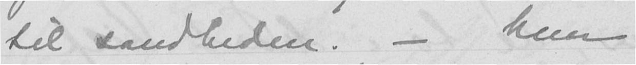til sandheden. - Men
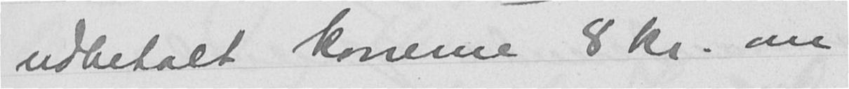udbetalt konerne 8 kr. om
